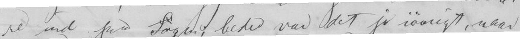re ind paa Sagen, bedre var det jo iøvrigt, naar
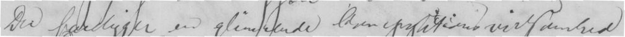Der foreligger en glimrende kompositions virksomhed
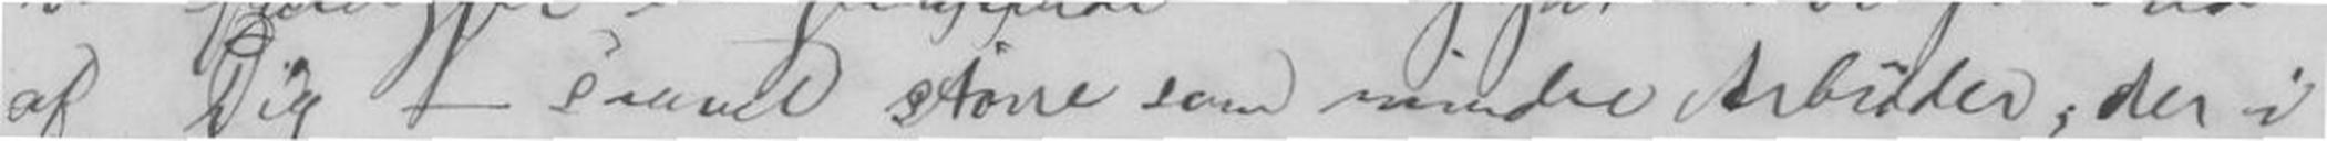af Dig - saavel større som mindre Arbeider, der i
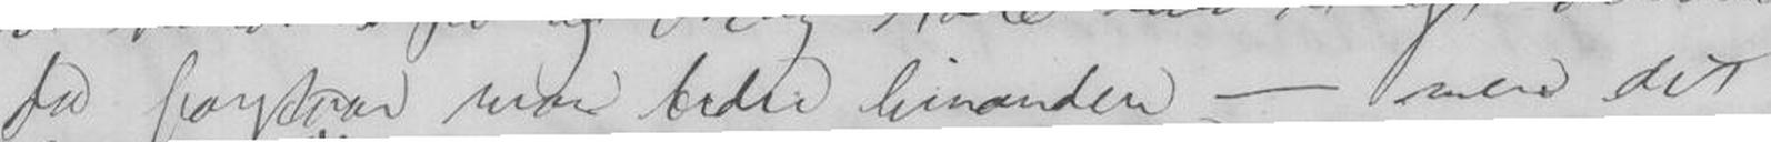da forstaar man bedre hinanden, - men det
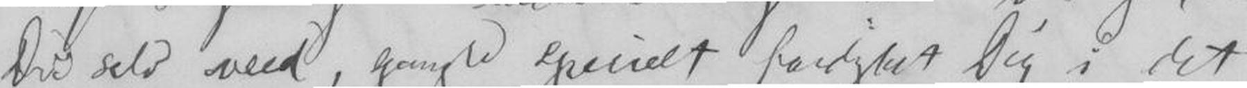Du selv veed, ganske specielt fordybet Dig i det
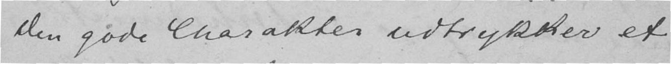Den gode Charakter udtrykker et
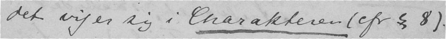det viger sig i Charakteren (cfr § 8).
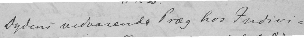Dydens vedvarende Præg hos Indivi-
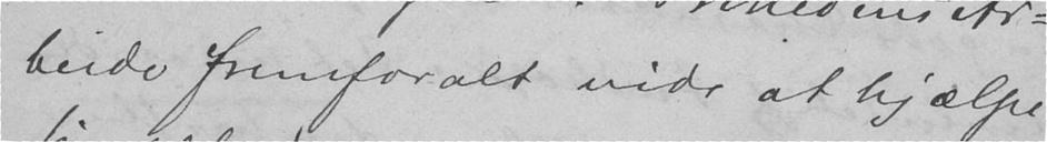beide fremforalt vide at hjælpe
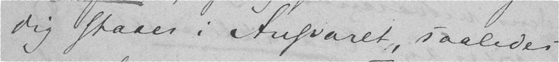dig staaer i Ansvaret, saaledes
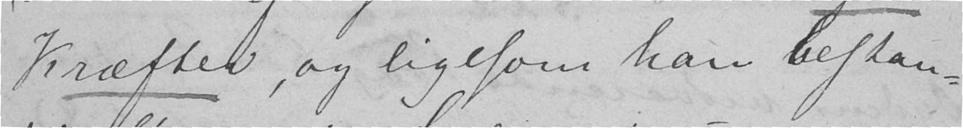Kræfter, og ligesom han bestan-
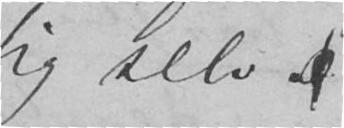sig selv.
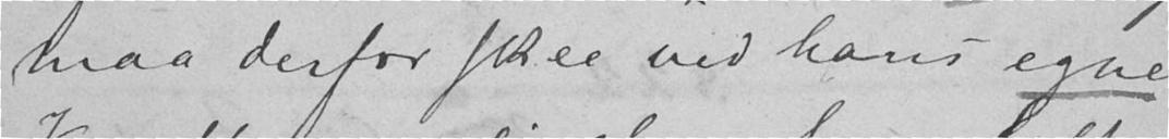maa derfor skee ved hans egne
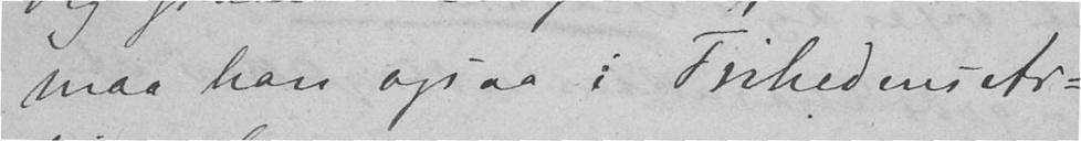maa han ogsaa i Frihedens Ar-
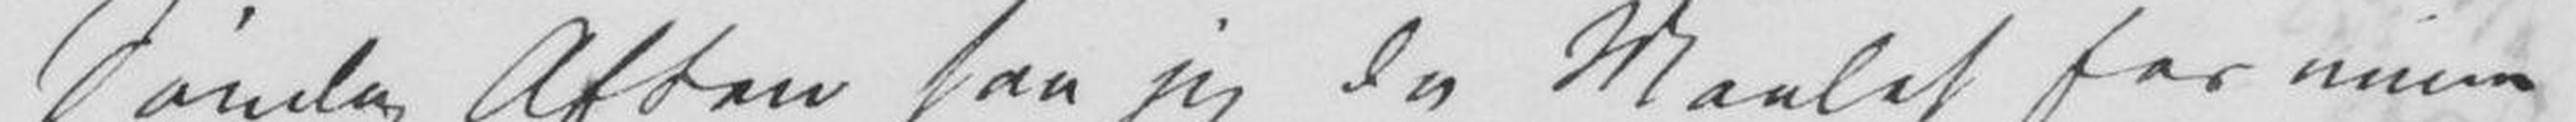Søndag Aften saa jeg da Maalet for mine
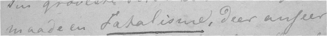maade en Fatalisme, der anseer
